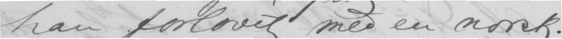han forlovet med en norsk.
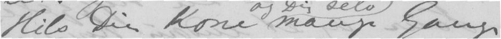baade fra min Kone
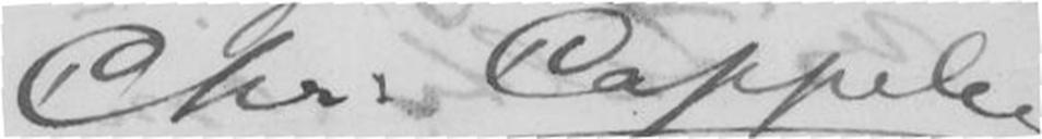Chr. Cappelen
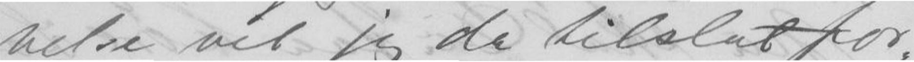velse vil jeg da tilslut for-
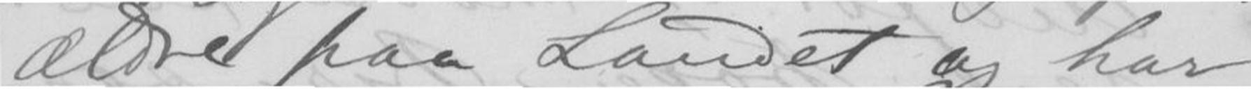ældre paa Landet og har
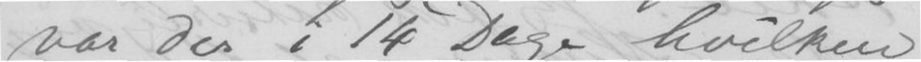var der i 14 Dage hvilken
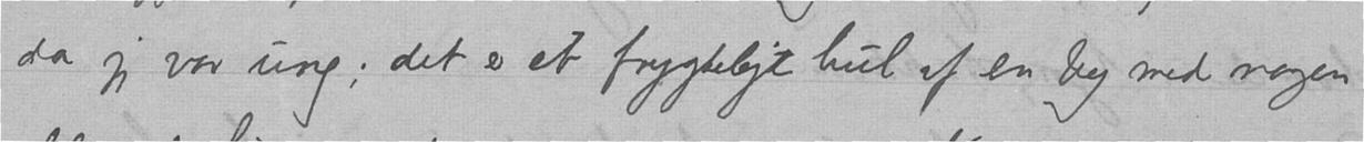da jeg var ung; det er et frygtelige hul af en by med nogen
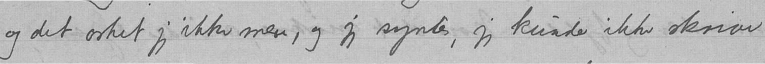og det orket jeg ikke mere, og jeg syntes, jeg kunde ikke skrive
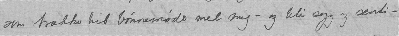som trækker til bønnemøder med mig - og blir syg og senti-
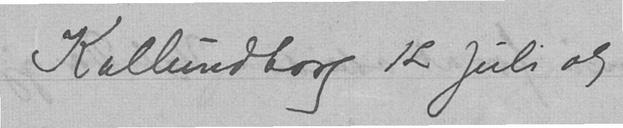Kallundborg 12. Juli 09
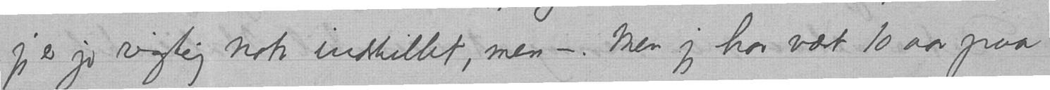jeg er jo rigtig nok indstillet, men -. Men jeg har vært 10 aar paa
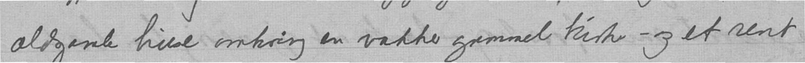ældgamle huse omkring en vakker gammel kirke - og et rent
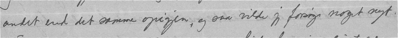andet end det samme opigjen, og saa vilde jeg forsøge noget nyt.
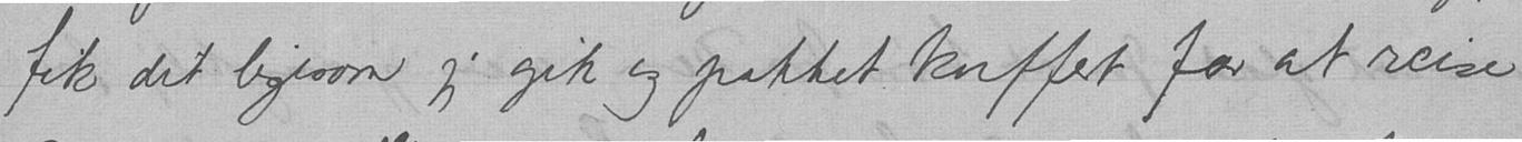fik det ligesom jeg gik og pakket kuffert for at reise
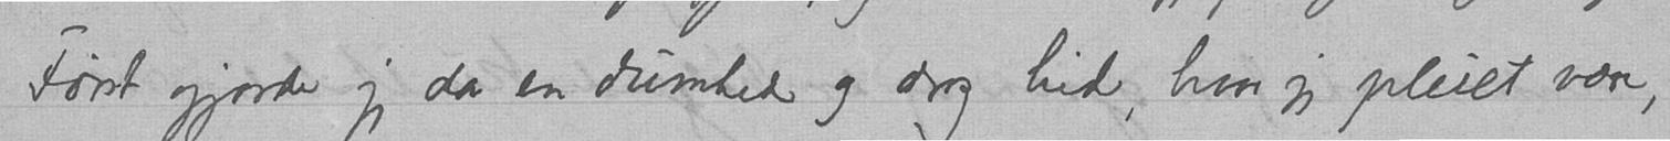Først gjorde jeg da en dumhed og drog hid, hvor jeg pleiet være,
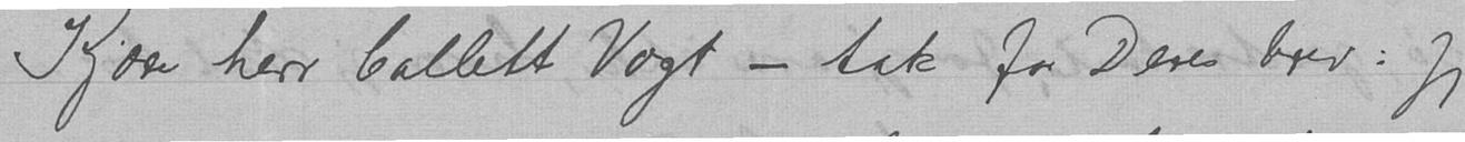Kjære herr Collett Vogt - tak for Deres brev: Jeg
